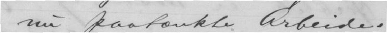nu paatænkte Arbeide.
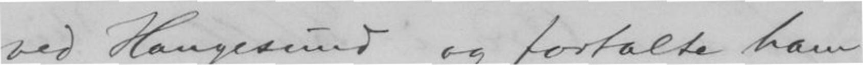ved Haugesund og fortalte ham
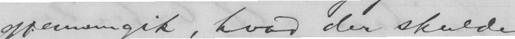gjennemgik, hvad der skulde
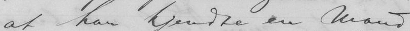at han kjendte en Mand
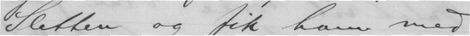Sletten og fik ham med
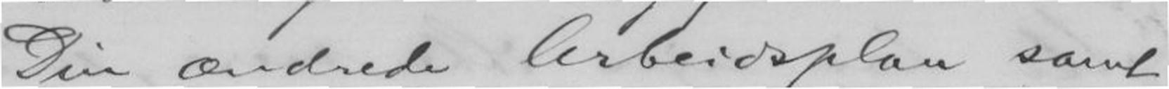Din ændrede Arbeidsplan samt
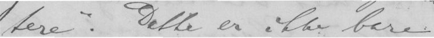tere". Dette er ikke bare
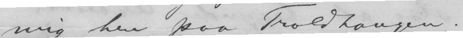mig hen paa Troldhaugen.
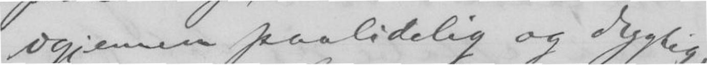igjennem paalidelig og dygtig,
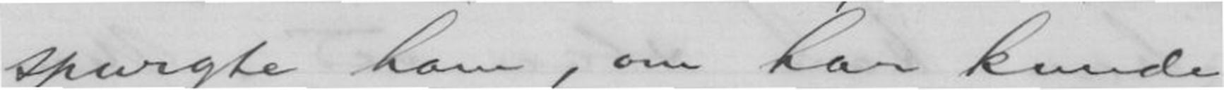spurgte ham, om han kunde
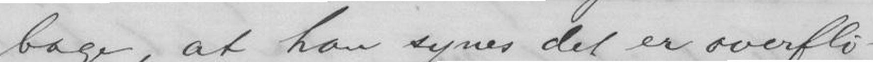bage, at han synes det er overflø-
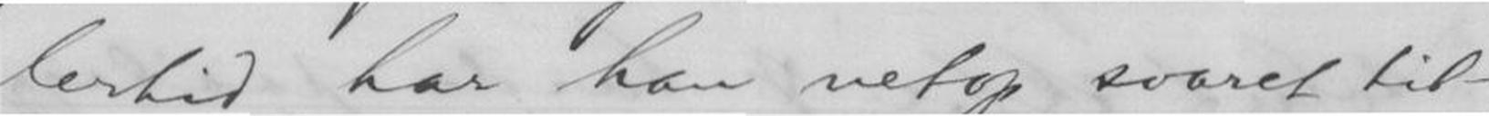lertid har han netop svaret til-
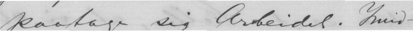paatage sig Arbeidet. Imid-
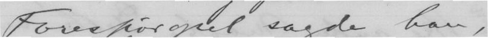Forespørgsel sagde han,
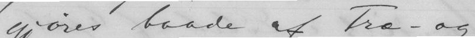gjøres baade af Træ- og
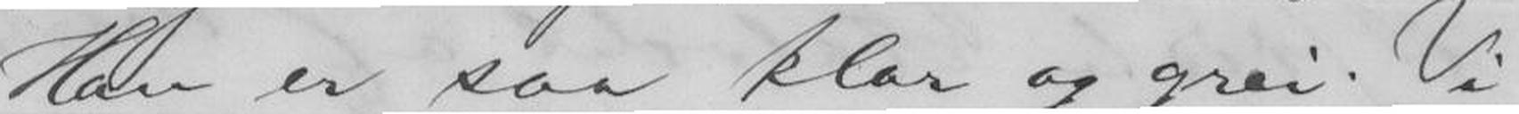Han er saa klar og grei. Vi
